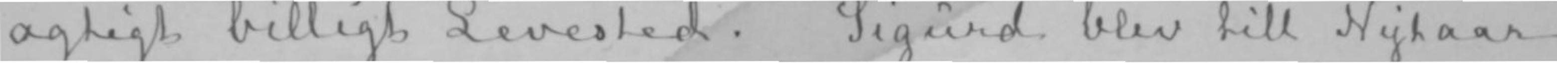agtigt billigt Levested. Sigurd blev till Nytaar
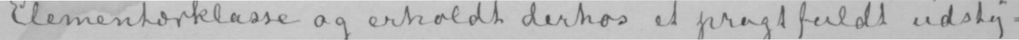Elementærklasse og erholdt derhos et pragtfuldt udsty-
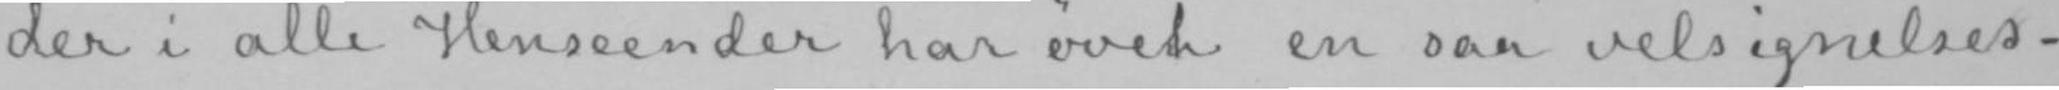der i alle Henseender har øvet en saa velsignelses-
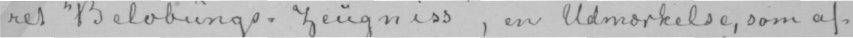ret «Belobungs-Zeugniss», en Udmærkelse, som af
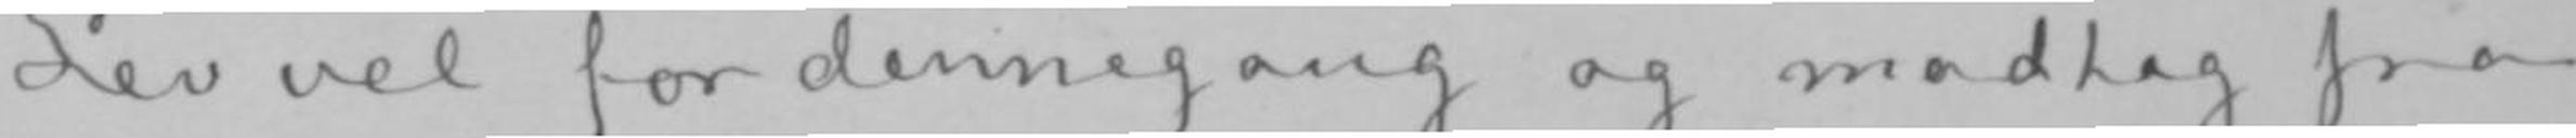Lev vel for dennegang og modtag fra
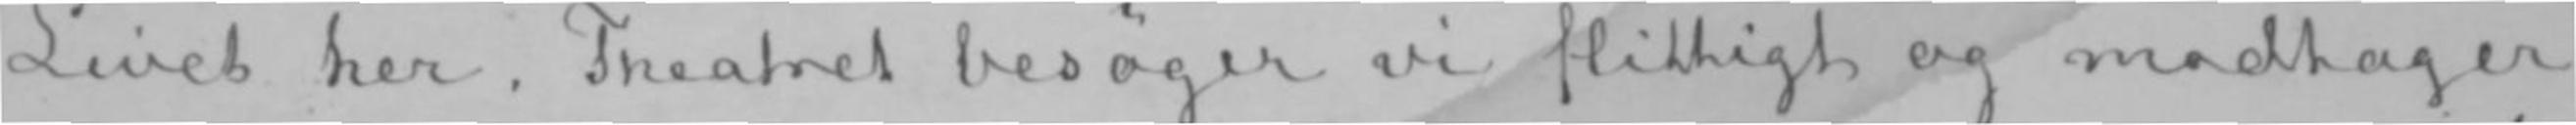Livet her. Theatret besøger vi flittigt og modtager
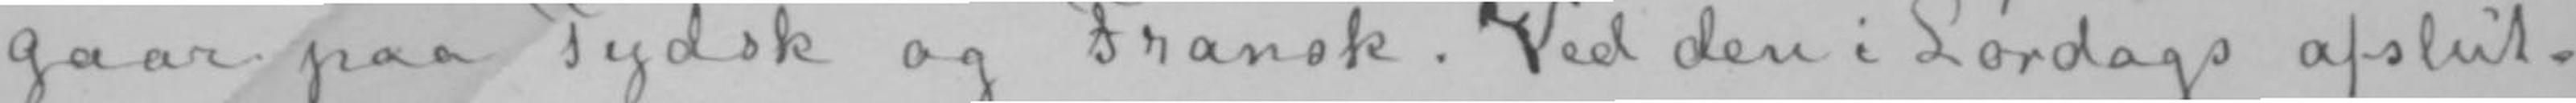gaar paa Tydsk og Fransk. Ved den i Lørdags afslut-
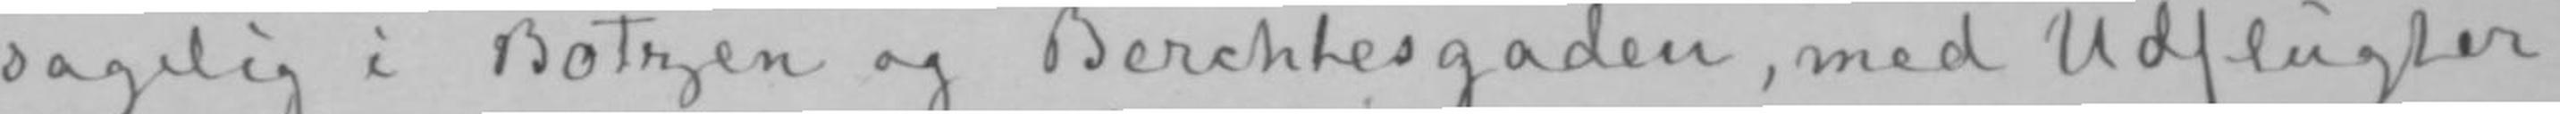sagelig i Botzen og Berchtesgaden, med Udflugter
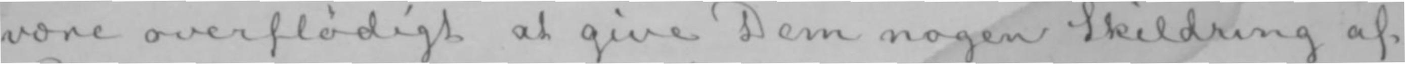være overflødigt at give Dem nogen Skildring af
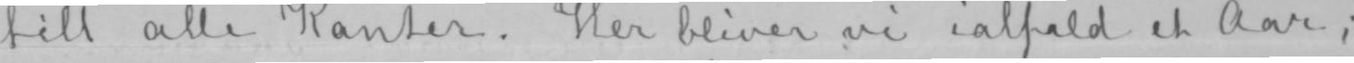till alle Kanter. Her bliver vi ialfald et Aar;
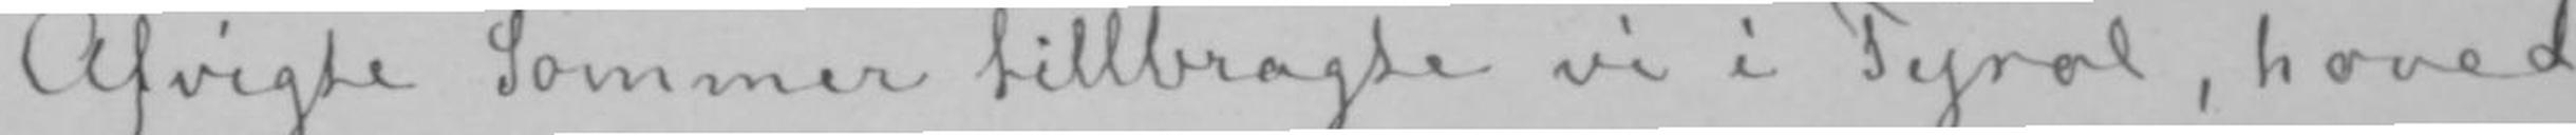Afvigte Sommer tillbragte vi i Tyrol, hoved-
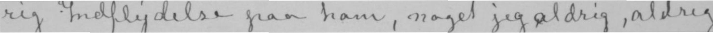rig Indflydelse paa ham, noget jeg aldrig, aldrig
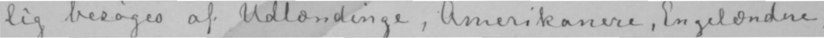lig besøges af Udlændinge, Amerikanere, Engelændre,
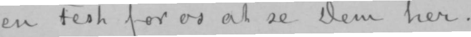en Fest for os at se Dem her.
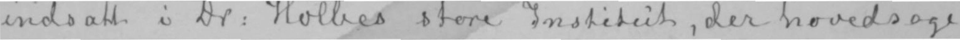indsatt i Dr: Hølbes store Institut, der hovedsage-
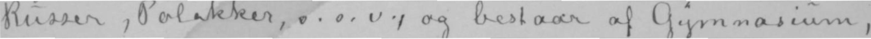Russer, Polakker, o. s. v., og bestaar af Gymnasium,
